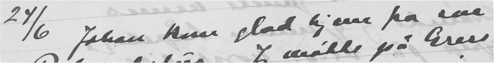24/6 Johan kom glad hjem fra sin
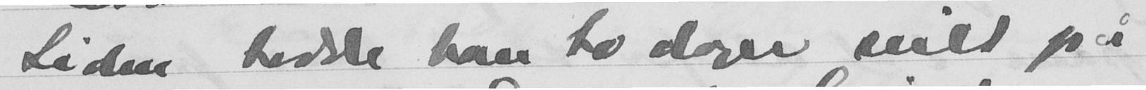Siden hadde han to dager seilt på
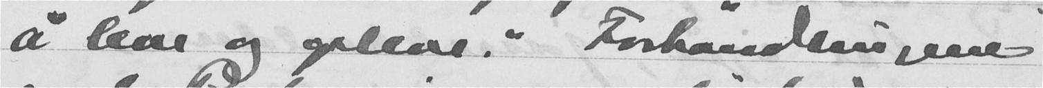å leve og opleve." Forhandlingene
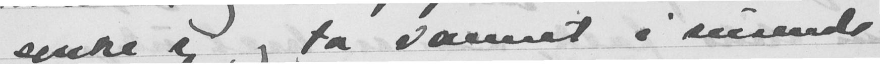senke sig og ta vannet i susende
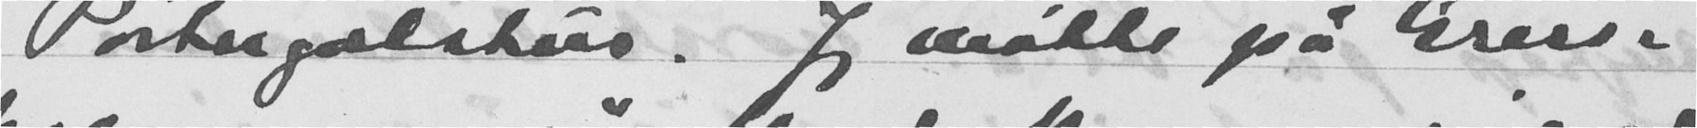Portugalstur. Jeg møtte på Gress-
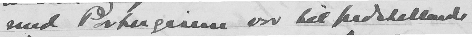med Portugiserne var tilfredstillende
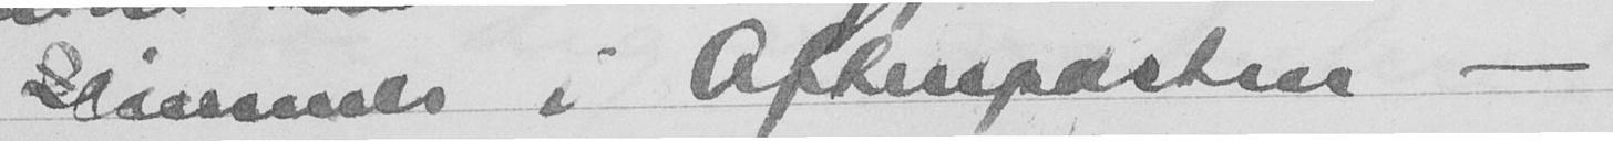Hausmes i Aftenposten -
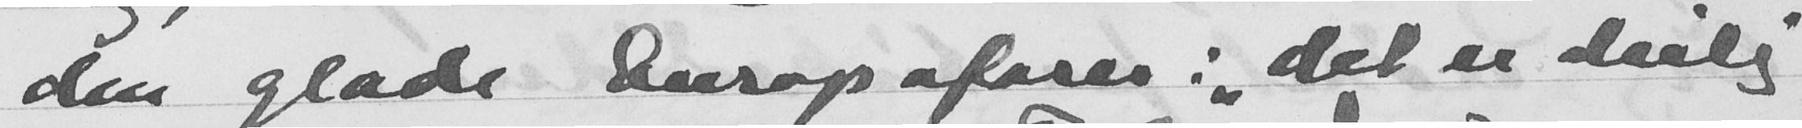den glade Europafarer: "det er deilig
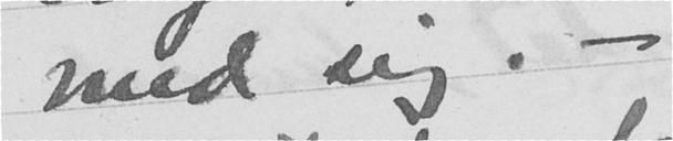med sig. -
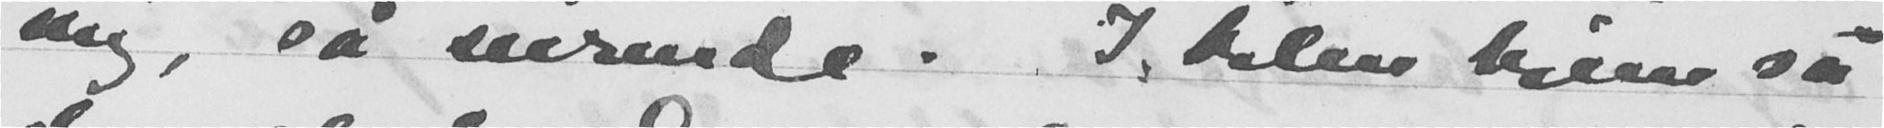mig, så srinde. I bilen hjem sa
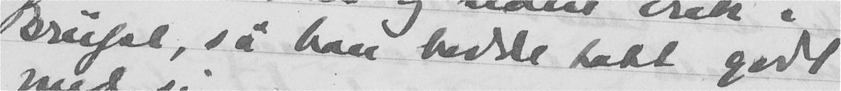Brüssel, så han hadde hatt godt
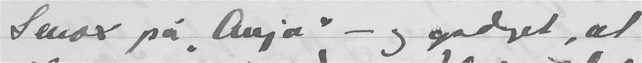Senoer på "Anja" - og opdaget, at
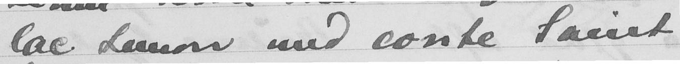lae Lemuor med cønte Taust
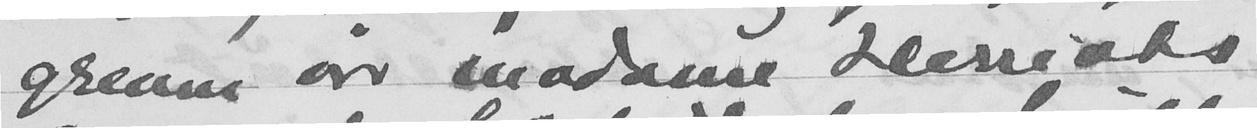greum var madame Hessiats
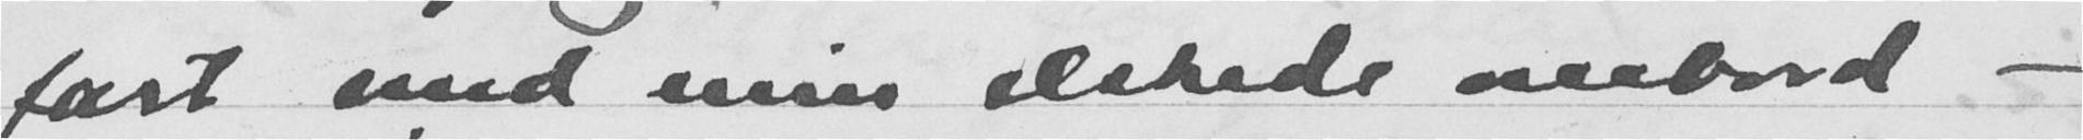fart med min elskede ombord -
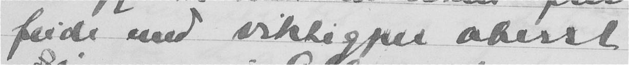feide med viktigper oberst
